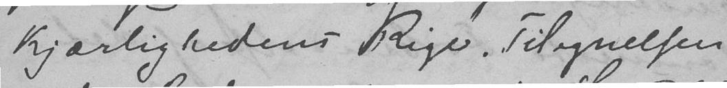Kjærlighedens Rige. Tilegnelsen
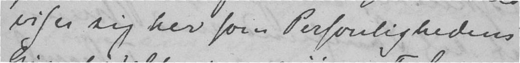viser sig her som Personlighedens
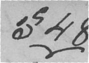§ 48
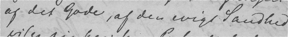af det Gode, af den evige Sandhed
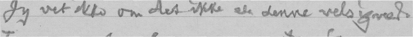Jeg vet ikke om det ikke er denne velsignede
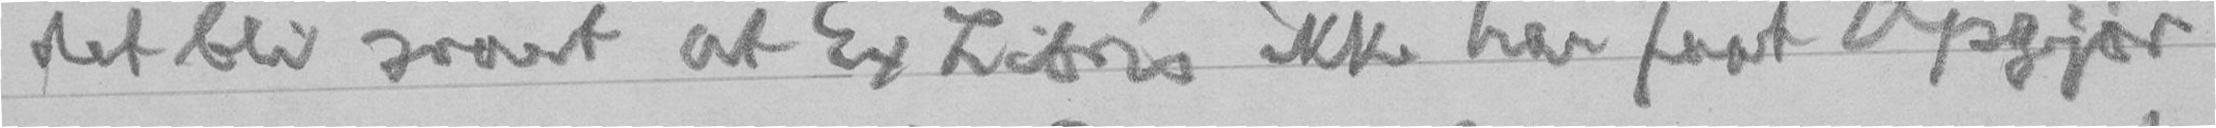det bli svart at Ex Libris ikke har faat Opgjør
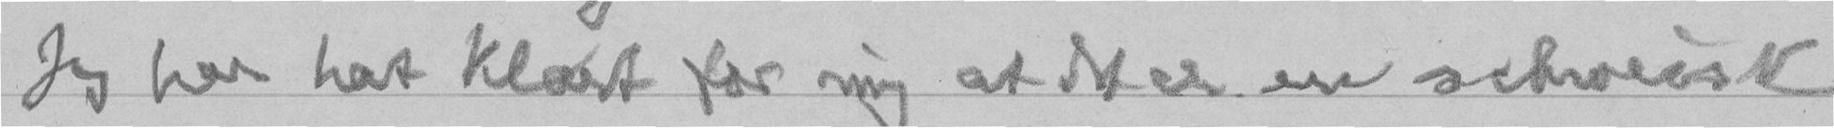Jeg har hat klart for mig at det er en sehveisk
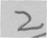2
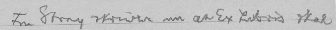Fru Stray skriver nu at Ex Libris skal
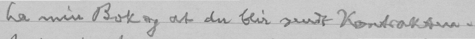ha min Bok og at du blir sendt Kontrakten.
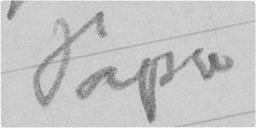Papa
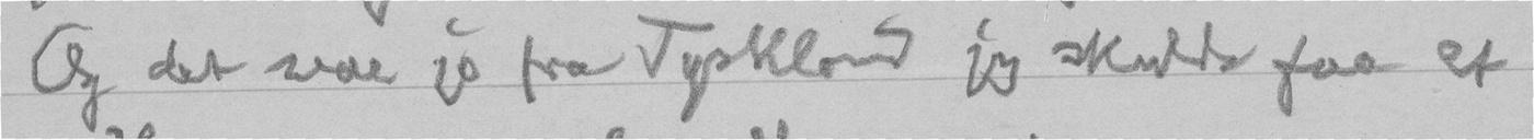Og det var jo fra Tyskland jeg skulde faa et
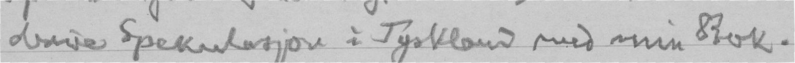drive Spekulasjon i Tyskland med min Bok.
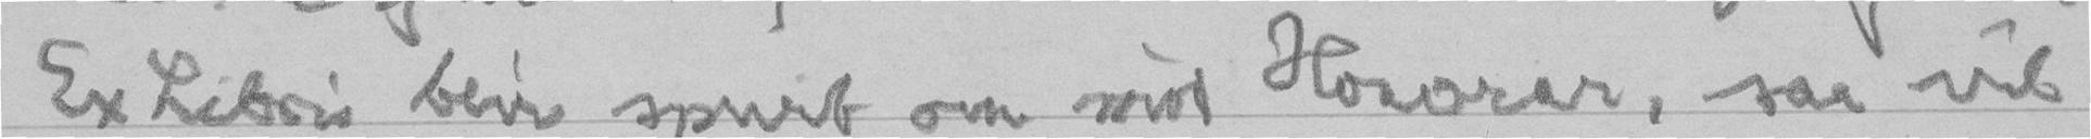Ex Libris blir spurt om mit Honorar, saa vil
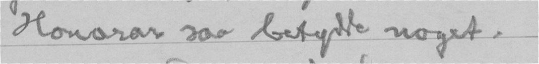Honorar saa betydde noget.
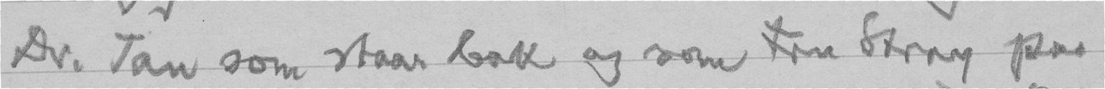Dr. Tau som staar bak og som Fru Stray paa
